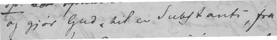og gjør Gud til en Substants, fra
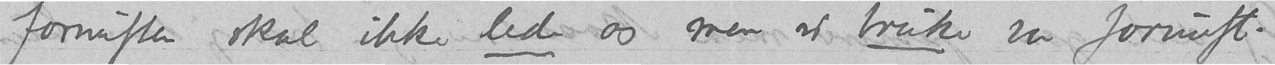fornuften skal ikke lede os men vi bruker vor fornuft.
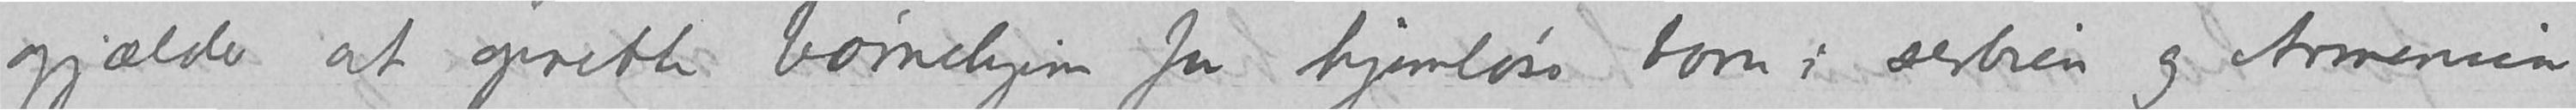gjælder at oprette børnehjem for hjemløse barn i Serbien og Armenien
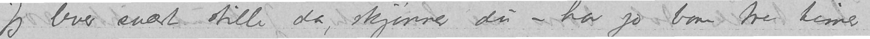Jeg lever svært stille da, skjønner du – har jo bare tre timer
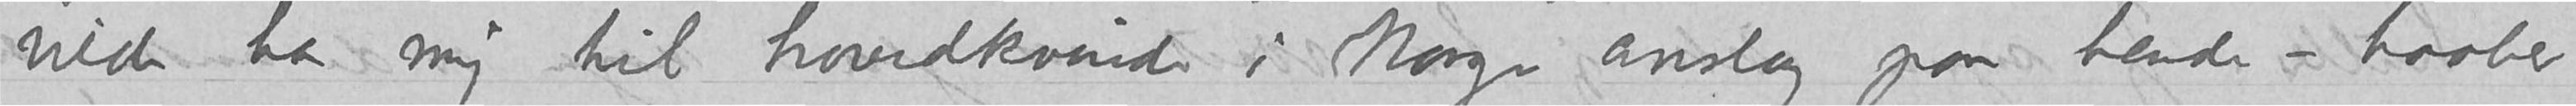vilde ha mig til hovedkvinde i Norge anslag paa hende – haaber
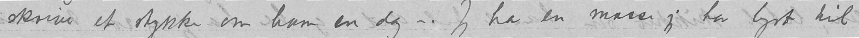skrive et stykke om ham en dag –. Jeg har en masse jeg har lyst til
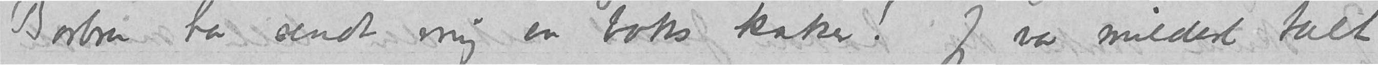Barbra har sendt mig en boks kaker! Jeg var mildest talt
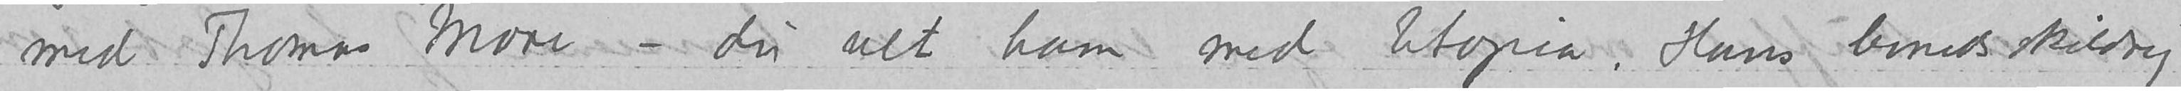med Thomas More – du vet ham med Utopia. Hans levnedsskildring
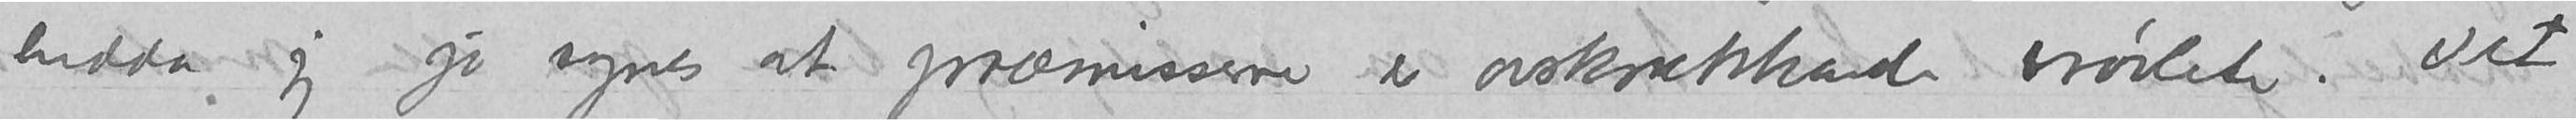endda jeg jo synes at præmisserne er avskrækkende vrøvlete. Det
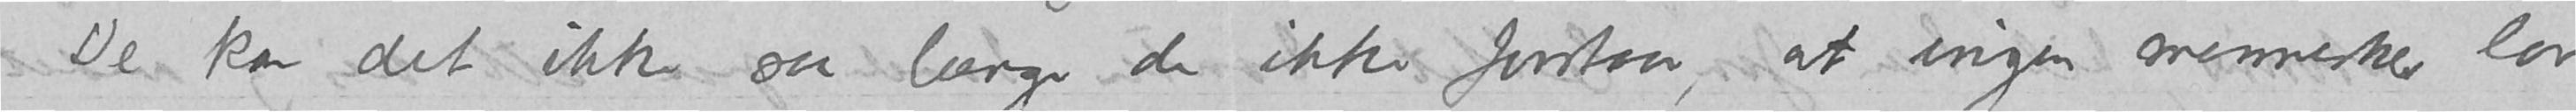De kan det ikke saa længe de ikke forstaar, at ingen menneske lar
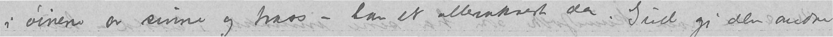i øinene av sinne og trass – han er allervakrest da. Gud gi den andre
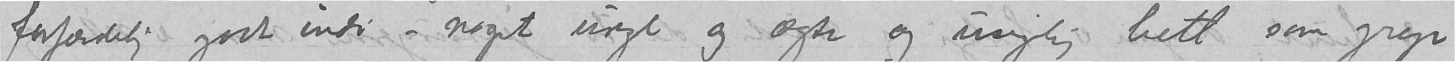forfærdelig godt indi – noget uægte og ægte og usigelig hett/lett som grep
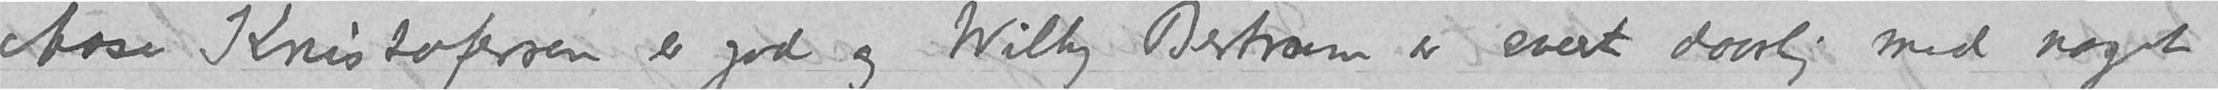Aase Kristofersen er god og Willy Bertram er svært daarlig med noget
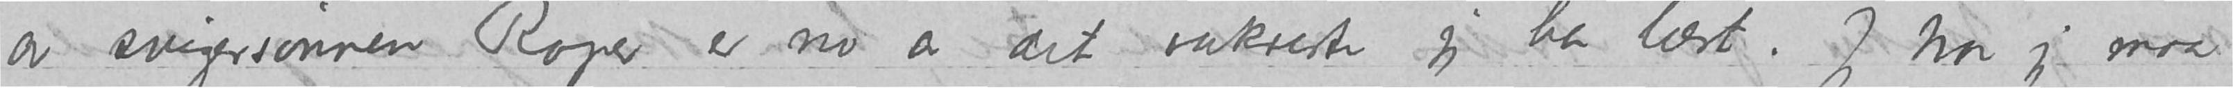av svigersønnen Roper er no av det vakreste jeg har læst. Jeg tror jeg maa
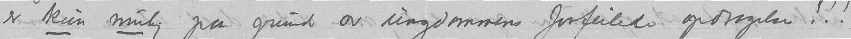er kun mulig paa grund av ungdommens forfeilede opdragelse!!!
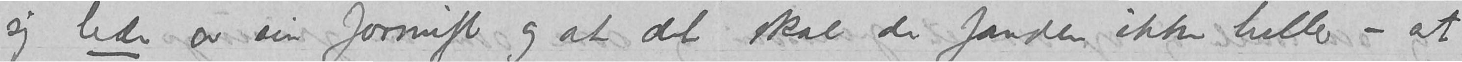sig lede av sin fornuft og at det skal de fanden ikke heller – at
